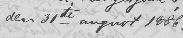den 31te august 1886.
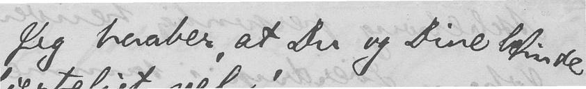Jeg haaber, at Du og Dine befinde
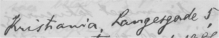Kristiania, Langesgade 5,
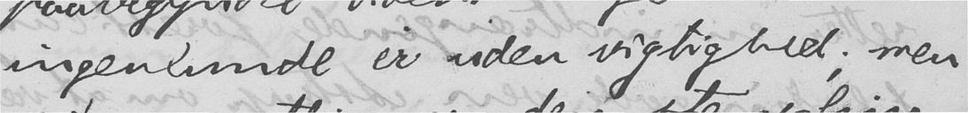ingenlunde er uden vigtighed; men
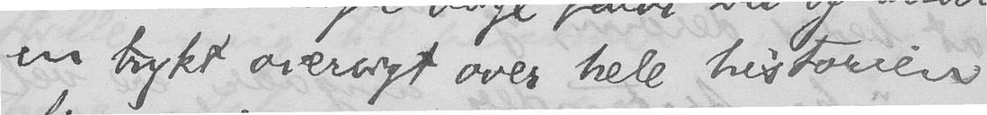en trykt oversigt over hele historien
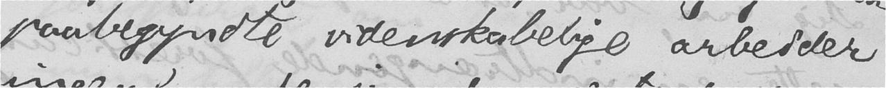paabegyndte videnskabelige arbeider
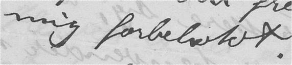mig forbeholdt.
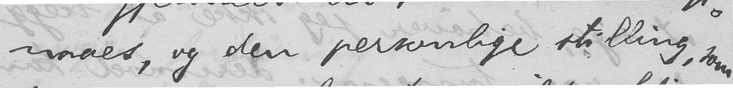naaes, og den personlige stilling, som
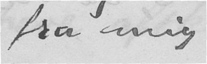fra mig.
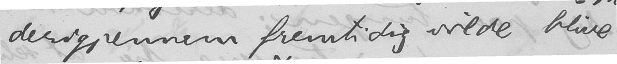derigjennem fremtidig vilde blive
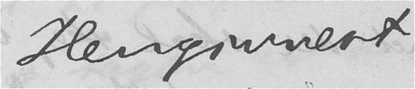Hengivnest
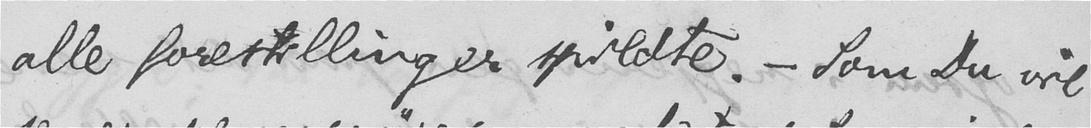alle forestillinger spildte. – Som Du vil
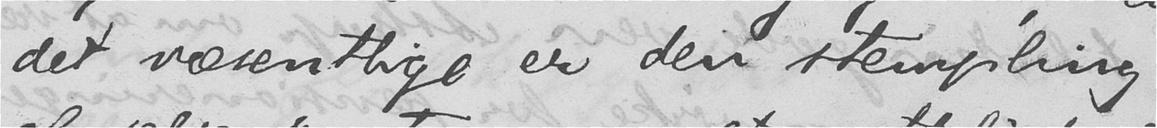det væsentlige er den stempling
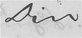Din
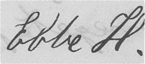Ebbe H.
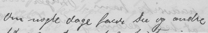Om nogle dage faar Du og andre
